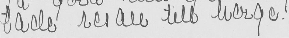OAlle bei Alb Kelb Nbr90.
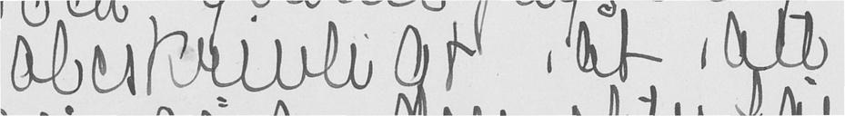Obeskrivlist iat sgld
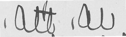Att Al
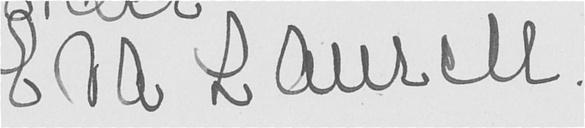3 106 L Allt el.
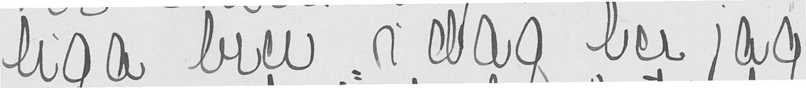lisa bese  200. Dei 1a4
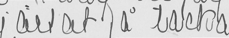Aldat 12 20cka.
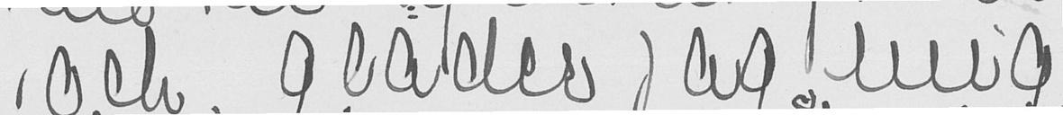Oet 9OAkleo 1A05 Uc00
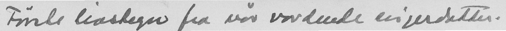Første livstegn fra vor vordende svigerdatter.
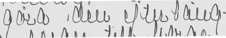90s Tlev ciet oAleG.
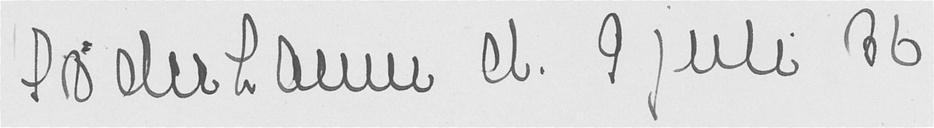SCcllb Callie Cb. 9jtilo 36
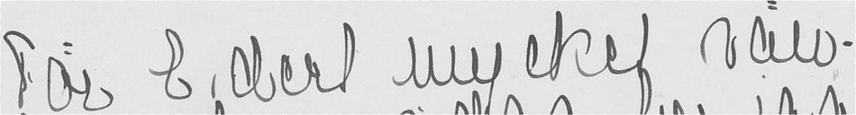T0l Eilberl Myckef belv.
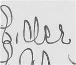Cillet
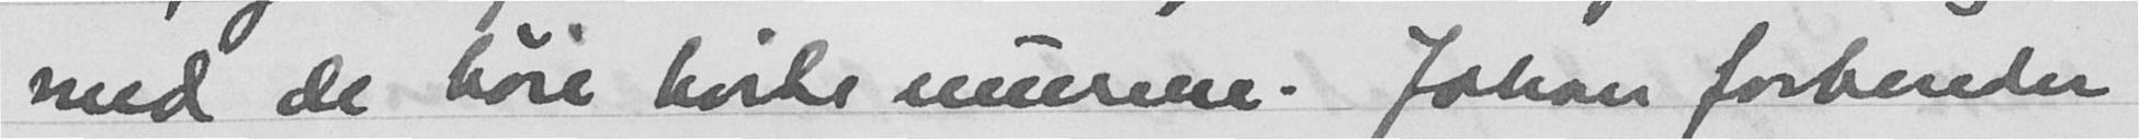med de høie hvite murene. Johan forbereder
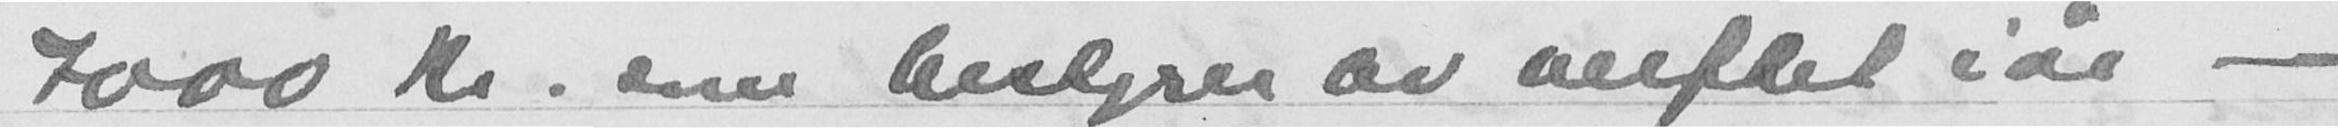4000 kr. som bestyrer av verftet iår -
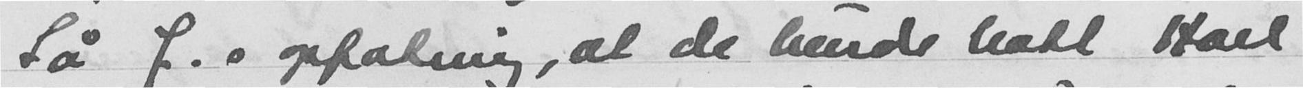Så, J.s opfatning, at de kunde hatt Hoel
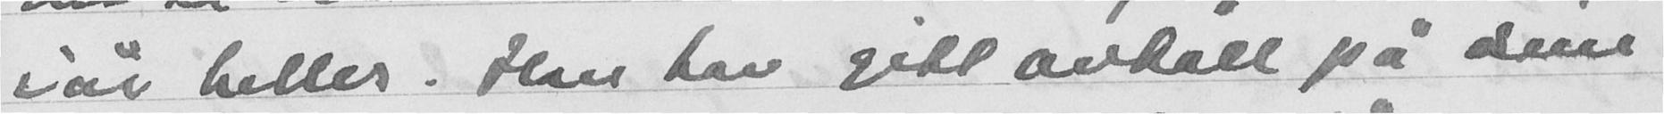i år heller. Han har gitt avkall på sine
